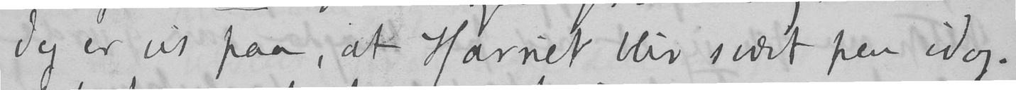Jeg er vis paa, at Harriet blir svært pen idag.
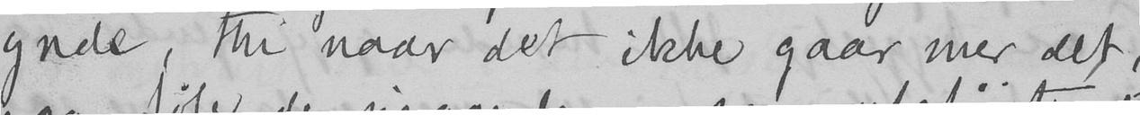ynde, thi naar det ikke gaar mer det,
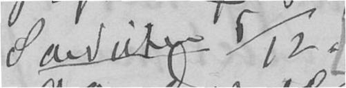Sandviken 5/12.
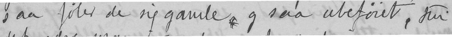saa føler de sig gamle, og saa ubeføiet, thi
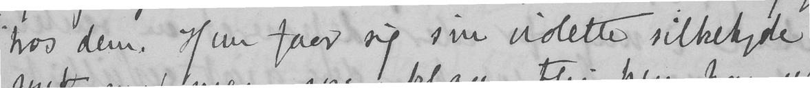hos dem. Hun faar sig sin violette silkekjole
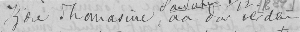Kjære Thomasine, aa du verden,
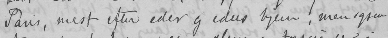Paris, mest efter eder og eders hjem, men igjen
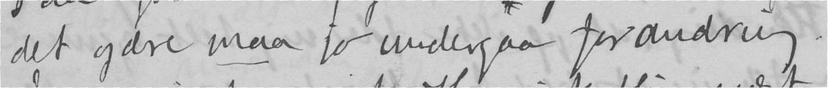det ydre maa jo undergaa forandring.
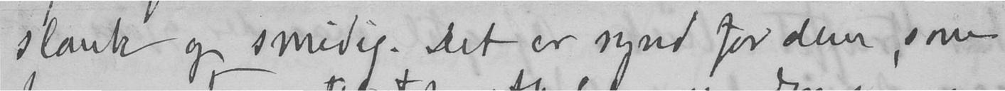slank og smidig. Det er synd for dem, som
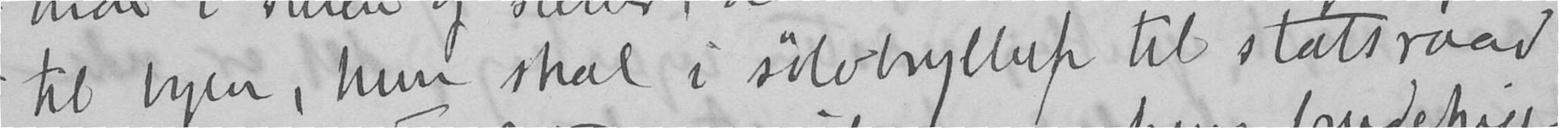til byen, hun skal i sølvbryllup til statsraad
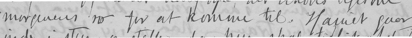morgenens ro for at komme til. Harriet gaar
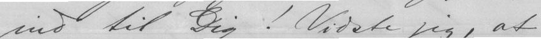ind til Dig! Vidste jeg, at
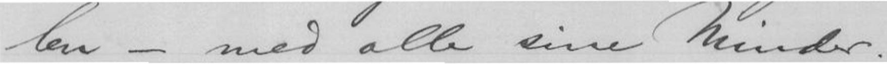len - med alle sine Minder.
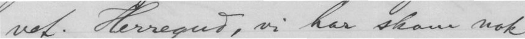vet. Herregud, vi har skam nok
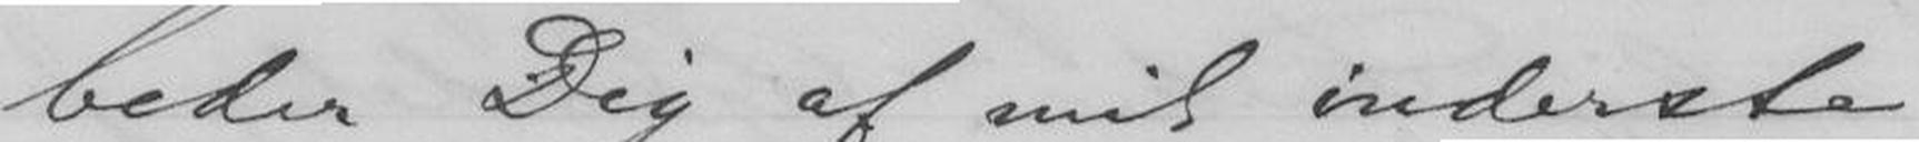beder Dig af mit inderste
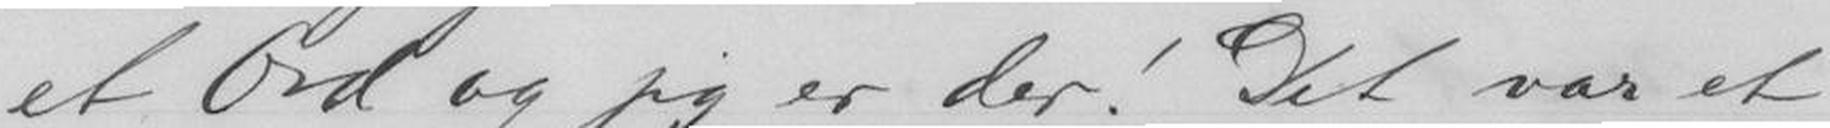et Ord og jeg er der! Det var et
